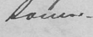Romer-
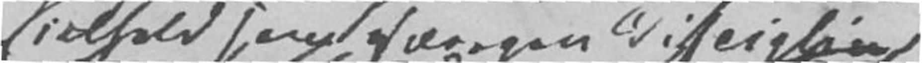ialfald som særegen Disciplin
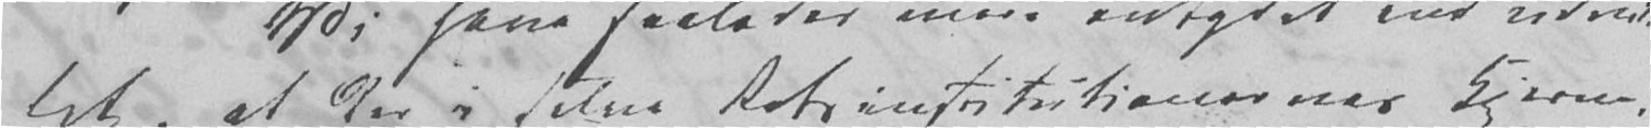let, at der i selve Retsinstitutionernes Kjerne,
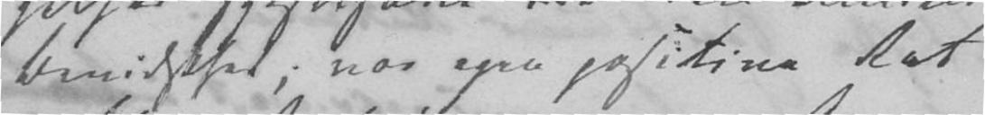Bevidshed; vor egne positive Ret
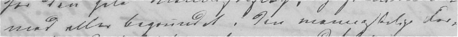med eller begrundet i den menneskelige For-
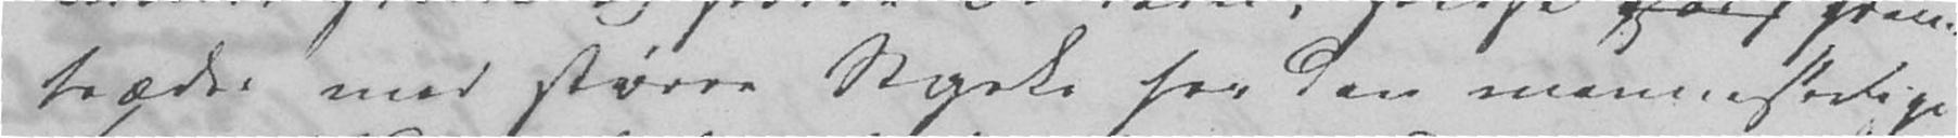træder med større Styrke for den menneskelige
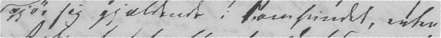gjør sig gjældende i Samfundet, enten
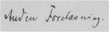Anden Forelæsning.
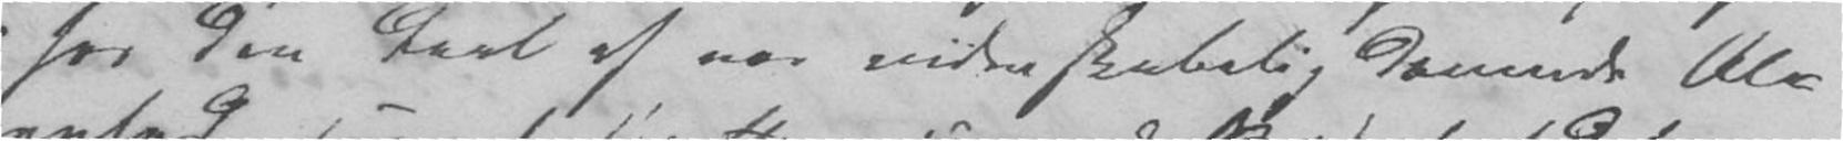hos den Deel at vor videnskabelig dannede Al-
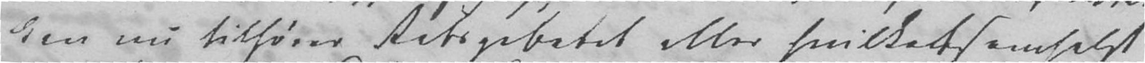den nu tilhører Retsgebetet eller hvilketsomhelst
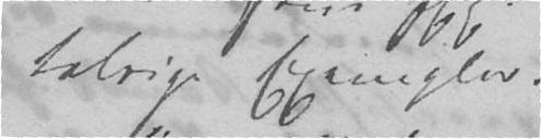talrige Exempler.
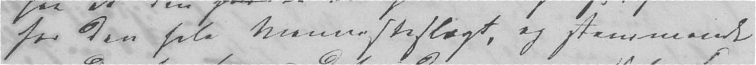for den hele Menneskeslægt, og stemmende
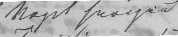Noget hvorpaa
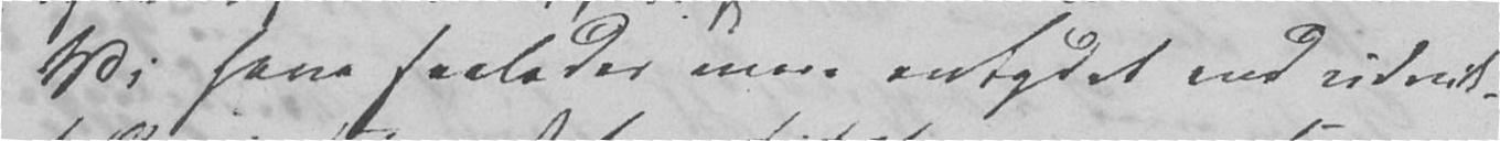Vi have saaledes mere antydet end udvik-
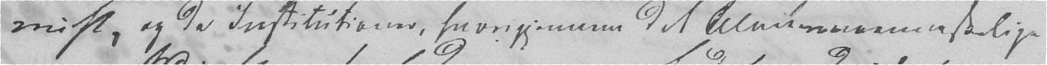nuft, og de Institutioner, hvorigjennem det Almeenmenneskelige
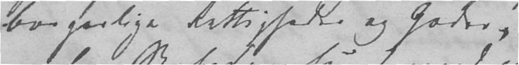borgerlige Rettigheder og Goder,
